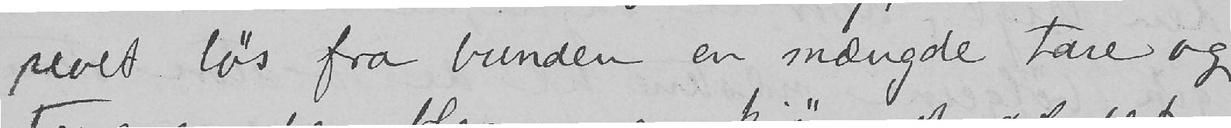revet løs fra bunden en mængde tare og
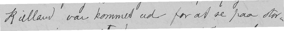Kielland var kommet ud for at se paa stor
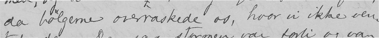da bølgerne overraskede os, hvor vi ikke ven-
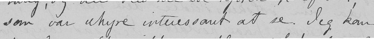som var uhyre interessant at se. Jeg kom
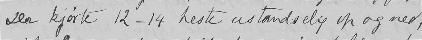Der kjørte 12–14 heste ustandselig op og ned,
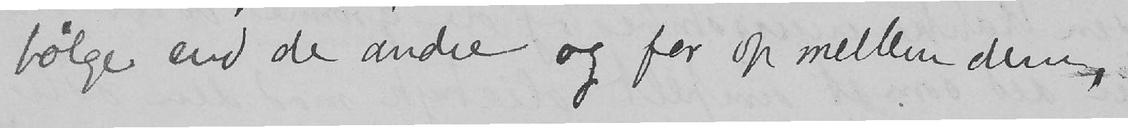bølge end de andre og for op mellem dem,
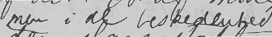men i al beskedenhed.
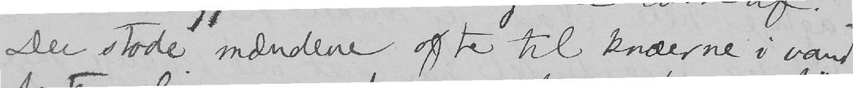Der stode mændene ofte til knæerne i vand
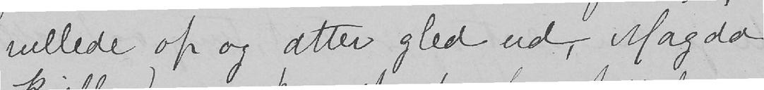rullede op og atter gled ud, Magda
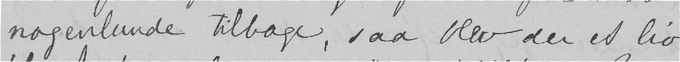nogenlunde tilbage, saa blev der et liv
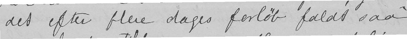det efter flere dages forløb faldt saa
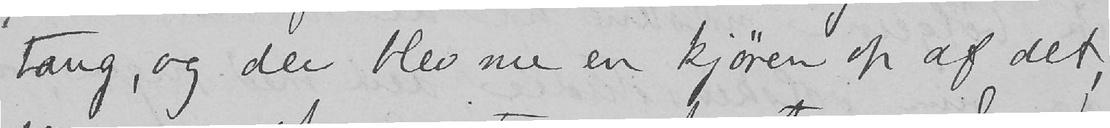tang, og der blev nu en kjøren op af det,
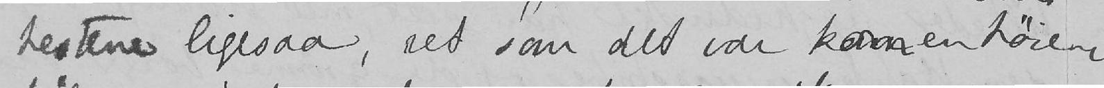hestene ligesaa, ret som det var kom en høiere
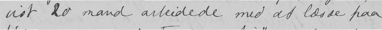vist 20 mand arbeidede med at læsse paa
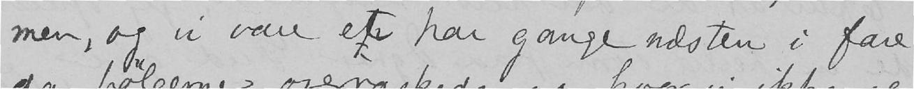men, og vi vare et par gange næsten i fare,
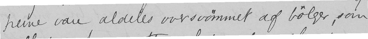perne vare aldeles oversvømmet af bølger, som
